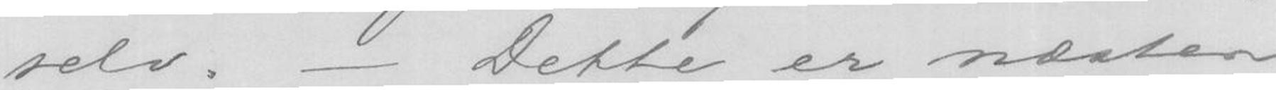selv.- Dette er næsten
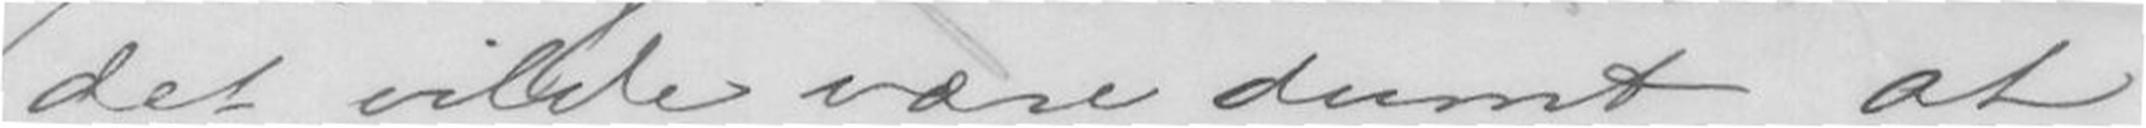det vilde være dumt at
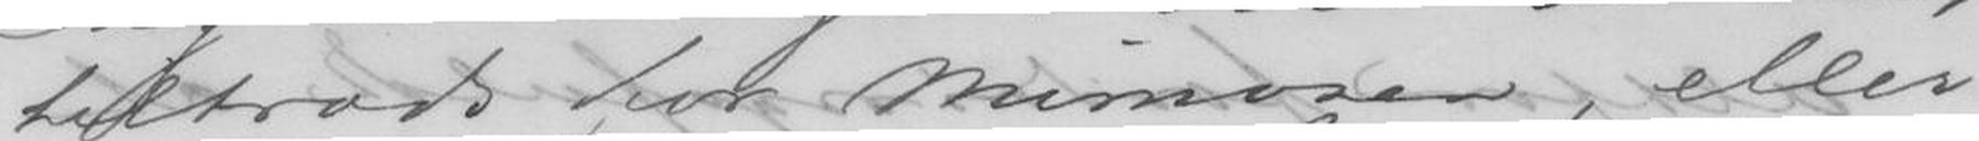tiltrods for Mimosen, eller
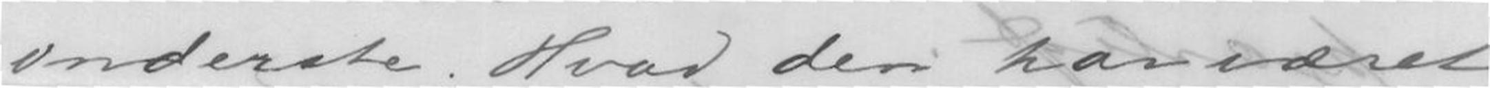inderste. Hvad den har været
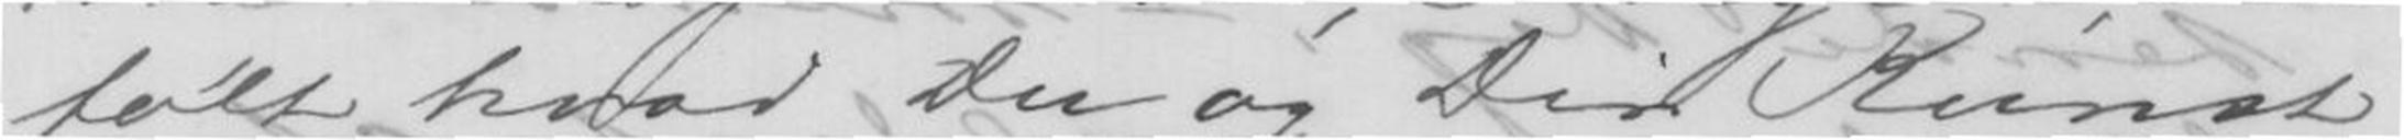følt hvad Du og Din Kunst
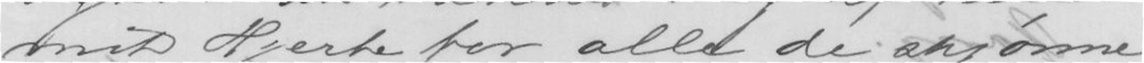mit Hjerte for alle de skjønne
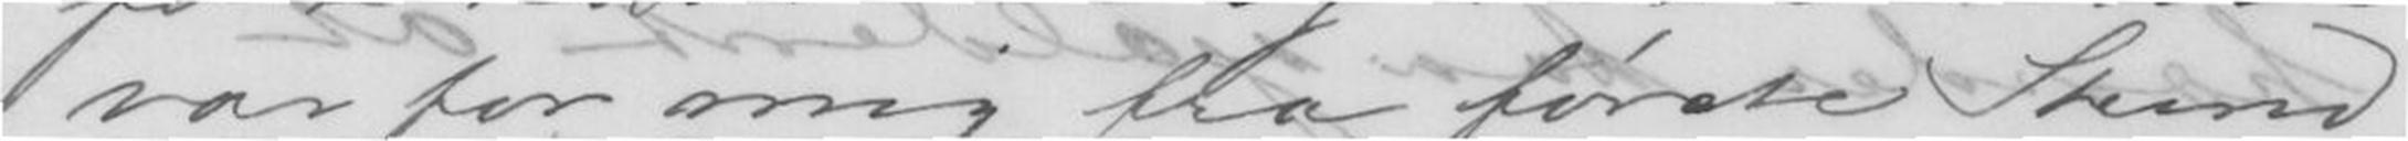var for mig fra første Stund,
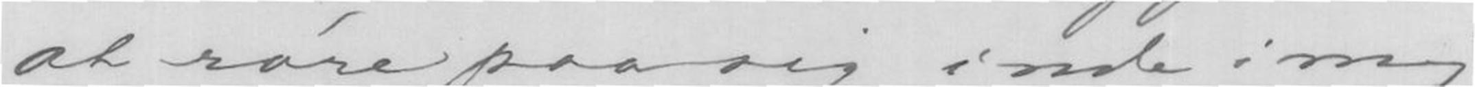at røre paa sig inde i mig
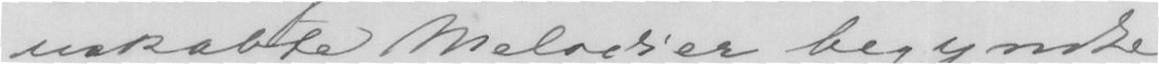uskabte Melodier begyndte
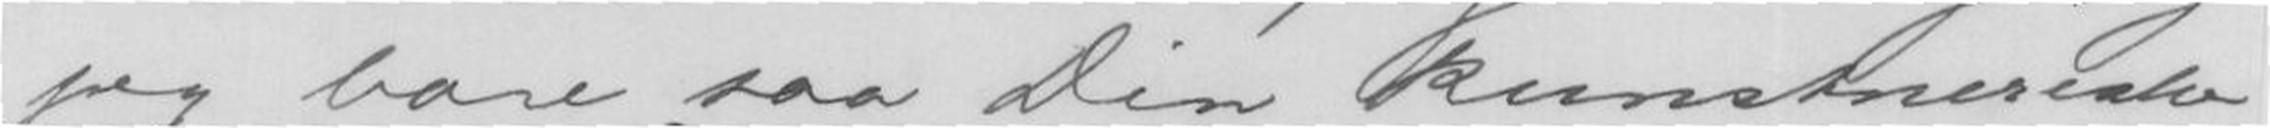jeg bare saa Din kunstneriske
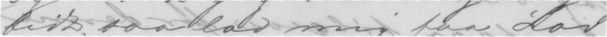lidt, saa lad mig faa Lov
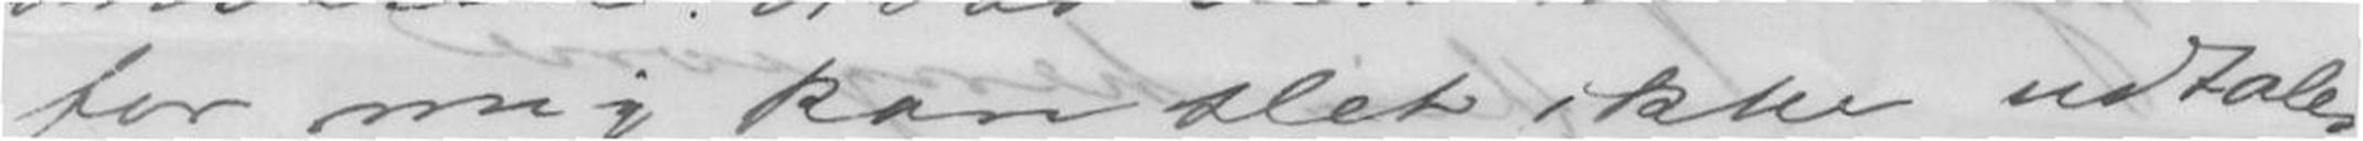for mig kan slet ikke udtales,
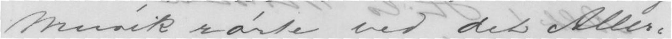Musik rørte ved det Aller-
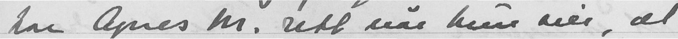har Agnes M. rett når hun sier, at
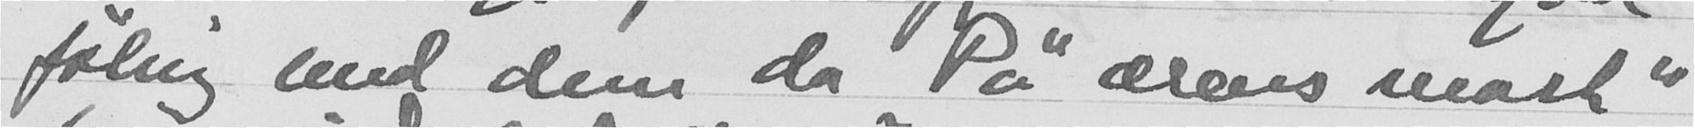føling med dem da "På ærens mark"
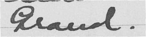Grand.
